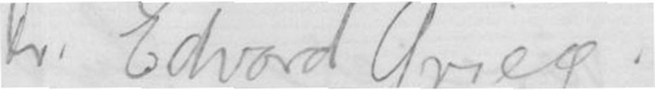hr Edvard Grieg.
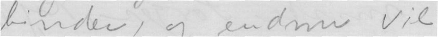binder, og endnu vil
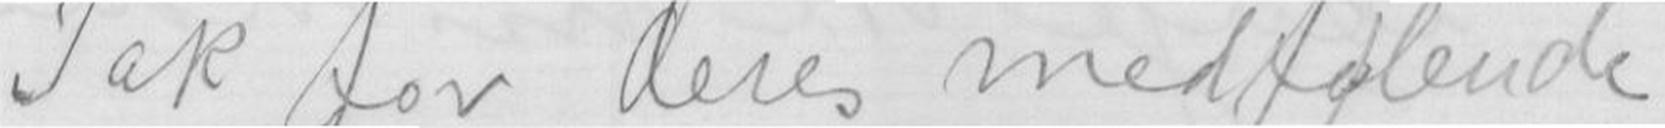Tak for Deres medfølgende
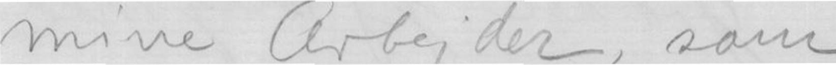mine Arbejder, som
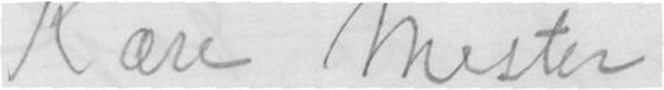Kære Mester
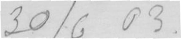30/6 03.
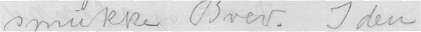smukke Brev. I den
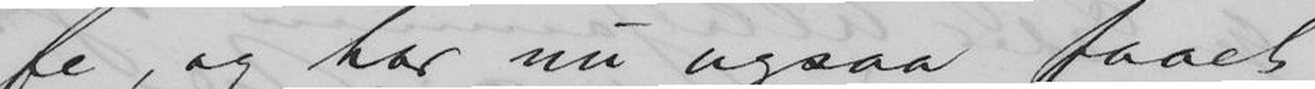fe, og har nu ogsaa faaet
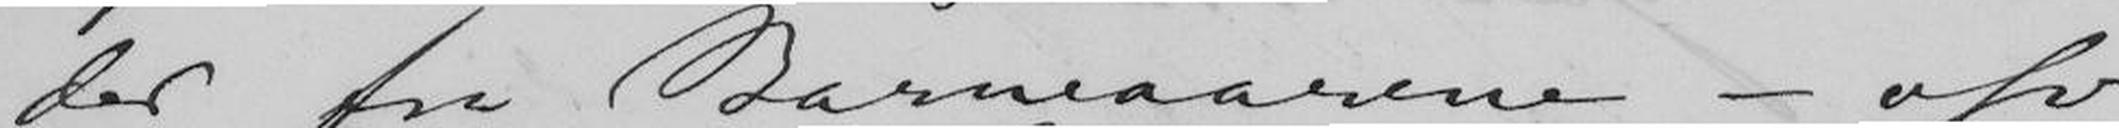der fra Barneaarene - osv
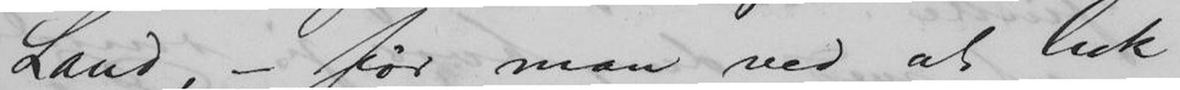Land, - før man ved at luk-
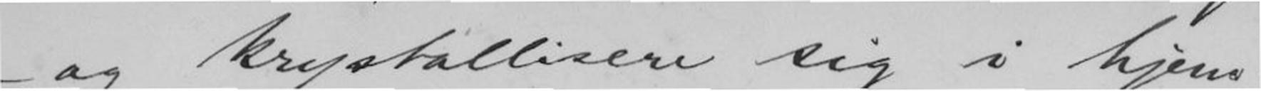- og krystallisere sig i hjem-
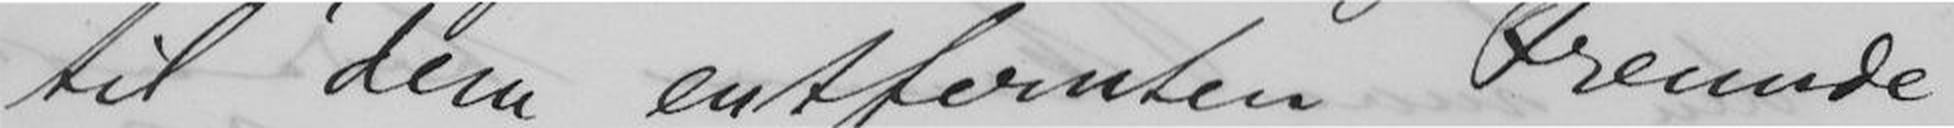til "dem entfernten Freunde",
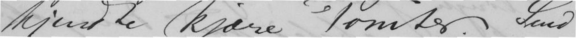kjendte kjære Tomter. Send
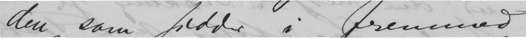den som sidder i fremmed
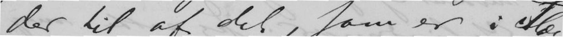der til af det, som er i Slægt
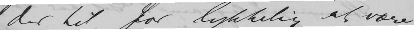der til for lykkelig at være!"
